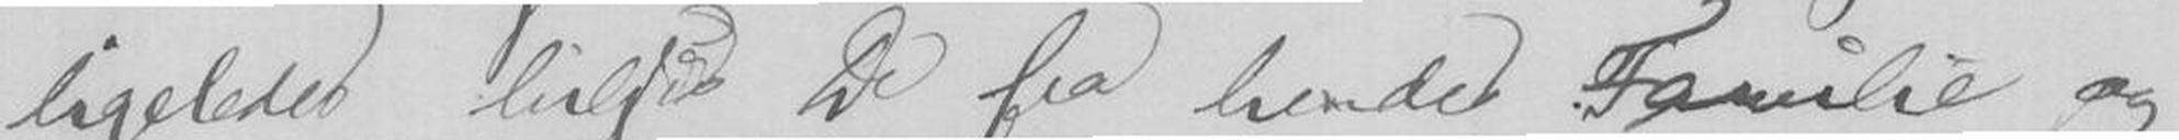ligelides hilser De fra hendes Familie og
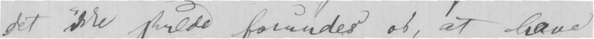det ikke skulde forundes os, at have
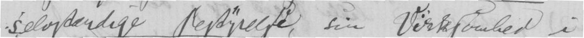selvstændige Bestyrelse, sin Virksomhed i
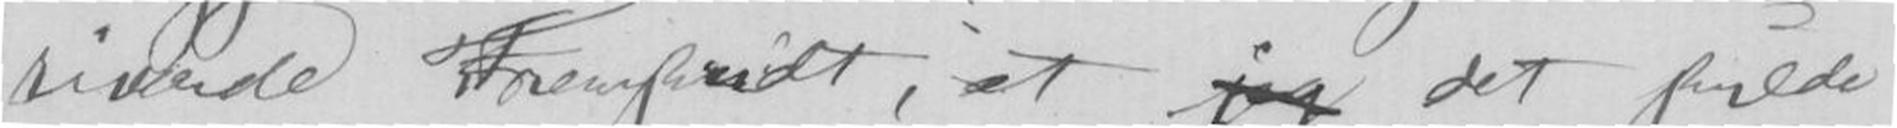rivende Fremskridt, at jeg det skulde
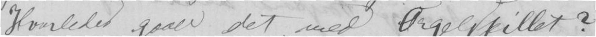Hvorledes gaar det med Orgelspillet?
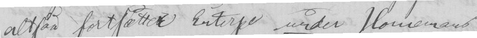altsaa fortsætter Euterpe under Hornemand
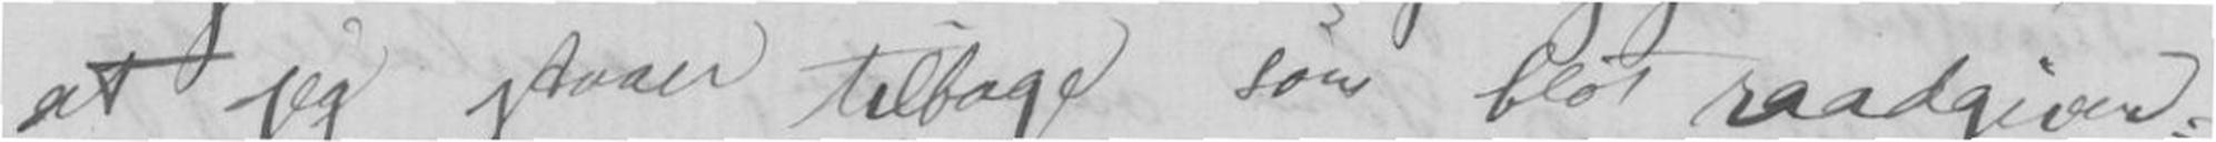at jeg svarer tilbage som blot raadgiver
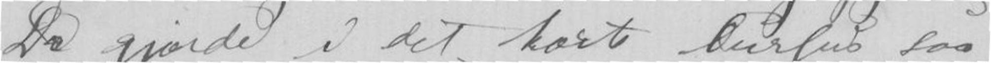Du gjorde i det korte Cursus saa
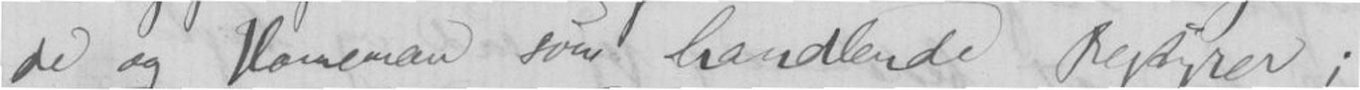de og Hornemand som handlende Bestyrer;
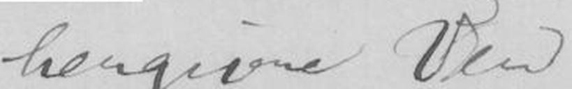hengivne Ven
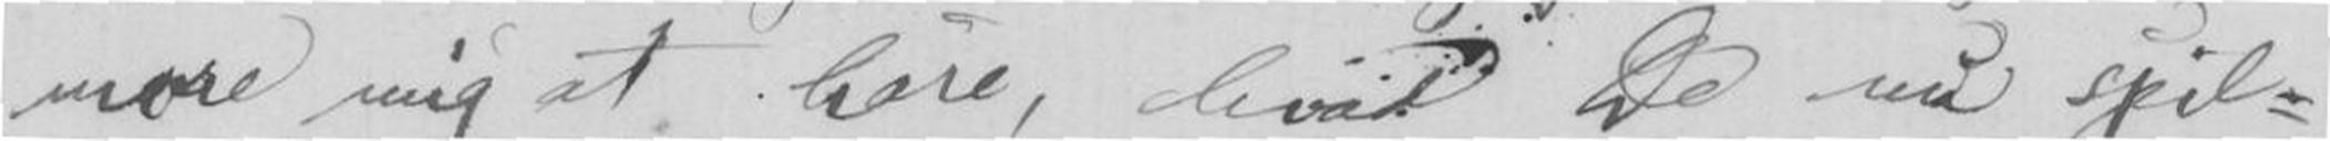more mig at høre, hvad De nu spil-
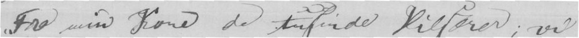"Fra min Kone de tusinde Hilsener; vi
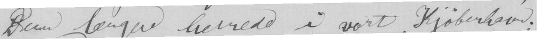Dem længere hernede i vort Kjøbenhavn,
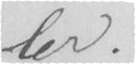ler.
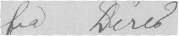fra Deres
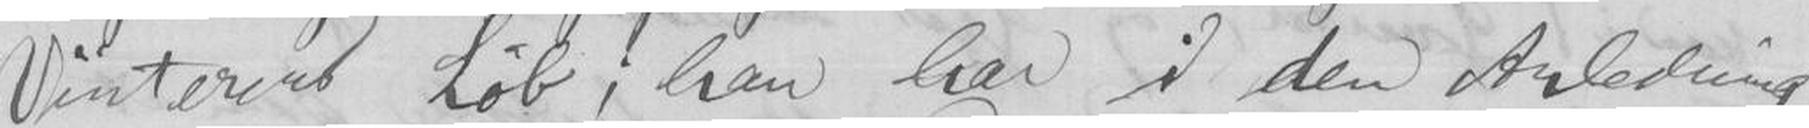Vinterens Løb; han har i den Anledning
